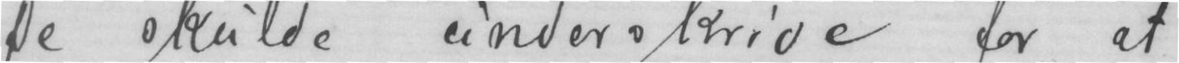De skulde underskrive for at
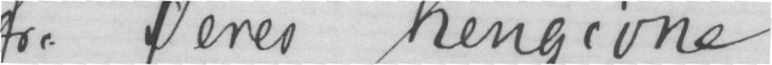fra Deres hengivne
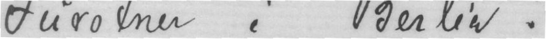Furotner i Berlin?
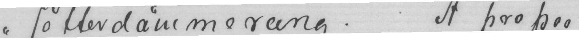Götterdämmerung. A propos
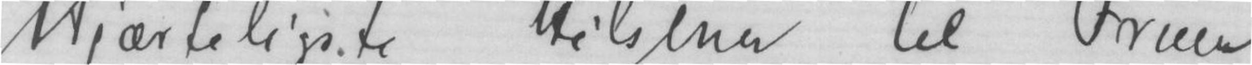Hjærteligste Hilsener til Fruen
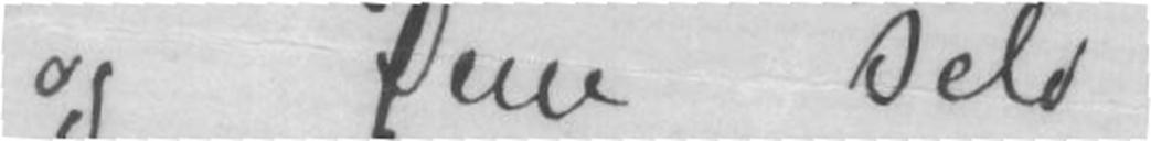og Dem selv
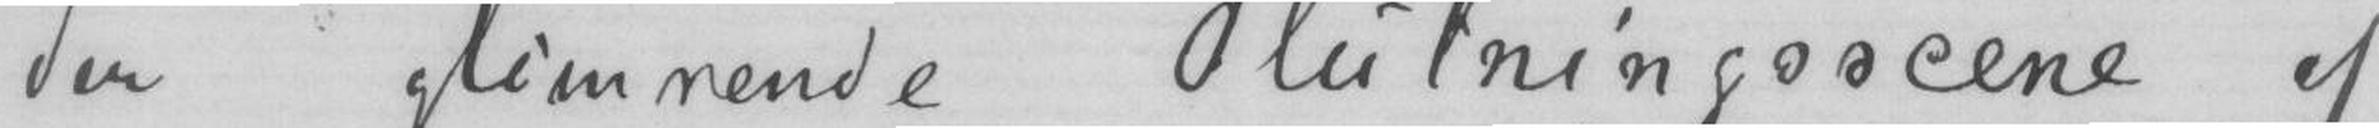den glimrende Slutningsscene af
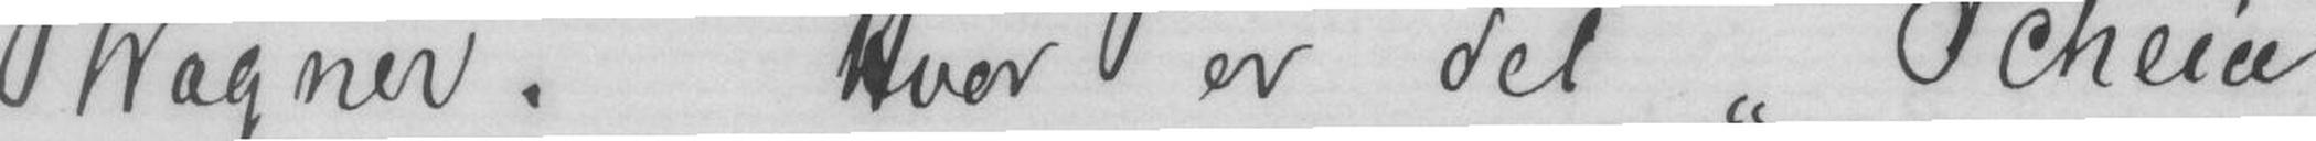Wagner. Hvor er det "Schein"
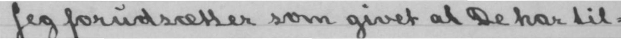Jeg forudsætter som givet at De har til-
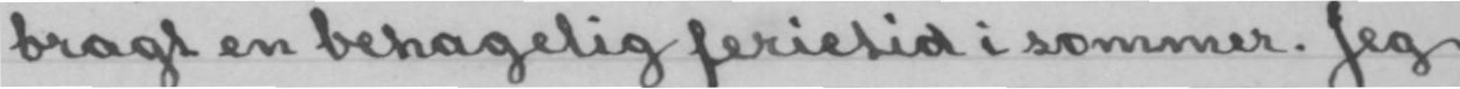bragt en behagelig ferietid i sommer. Jeg
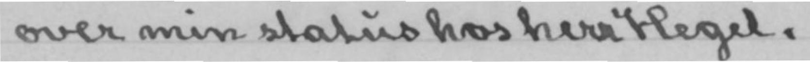over min status hos herr Hegel.
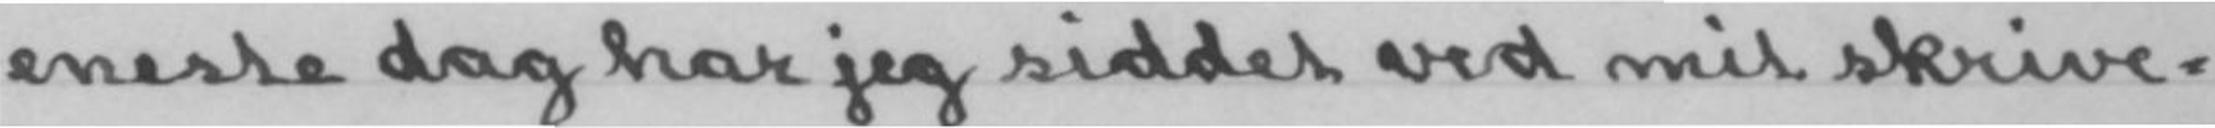eneste dag har jeg siddet ved mit skrive-
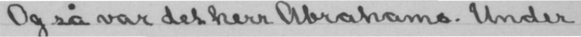Og så var det herr Abraham.s. Under
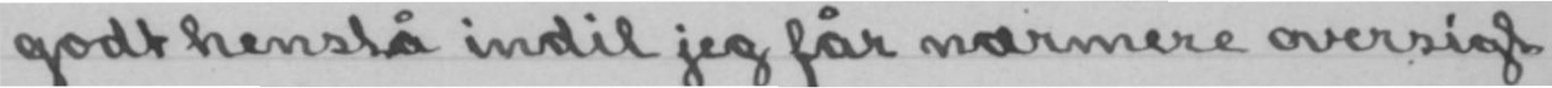godt henstå indil jeg får nærmere oversigt
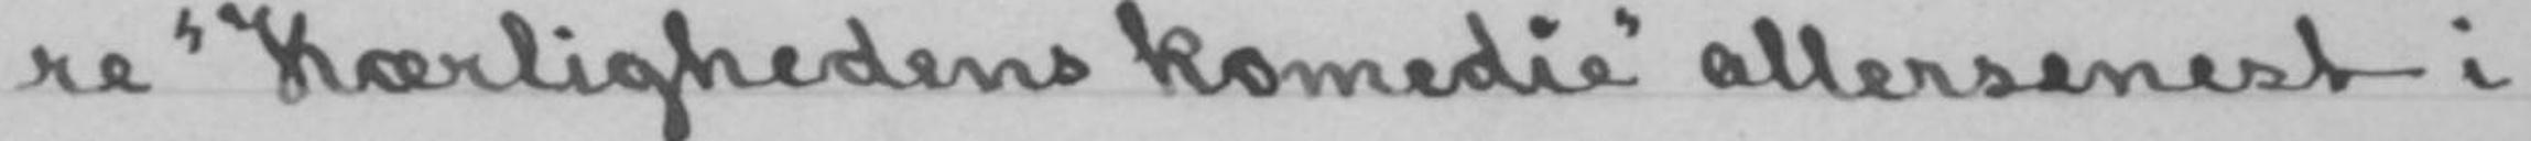re «Kærlighedens komedie» allersenest i
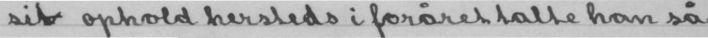sit ophold hersteds i foråret talte han så
